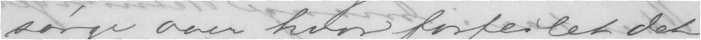sørge over hvor forfeilet det
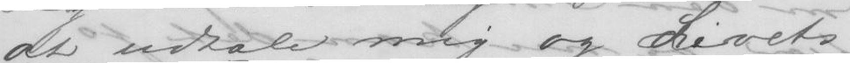at udtale mig og Livets
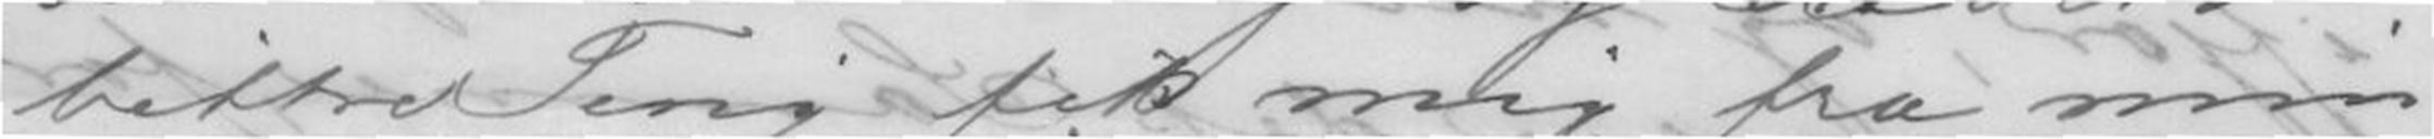bittre Ting fik mig fra min
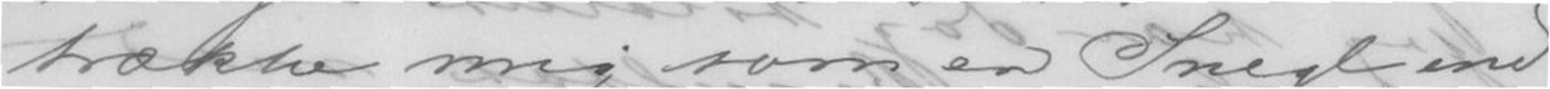trække mig som en Snegl ind
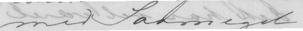med Saameget.
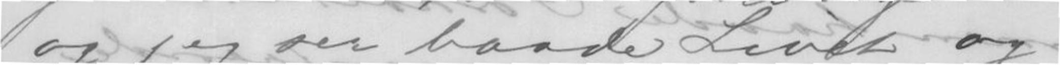og jeg ser baade Livet og
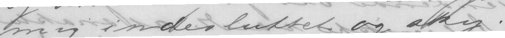mig indesluttet og sky.
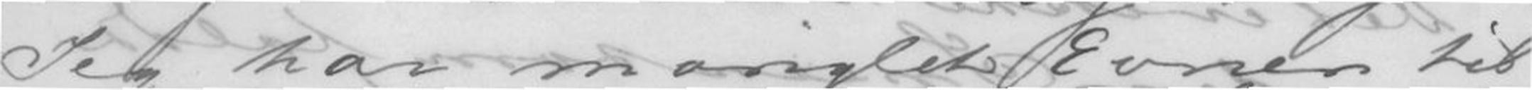Jeg har manglet Evnen til
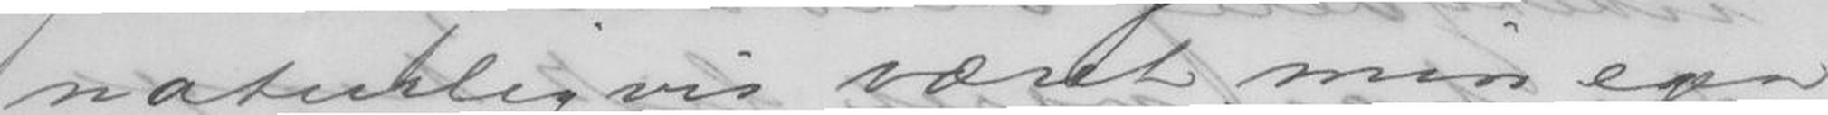naturligvis været min egen
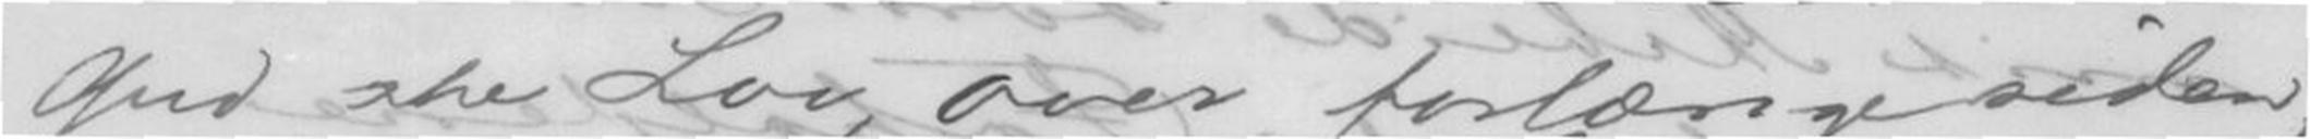Gud ske Lov, over forlængesiden,
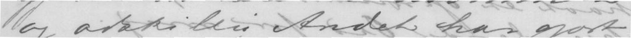og adskillig Andet har gjort
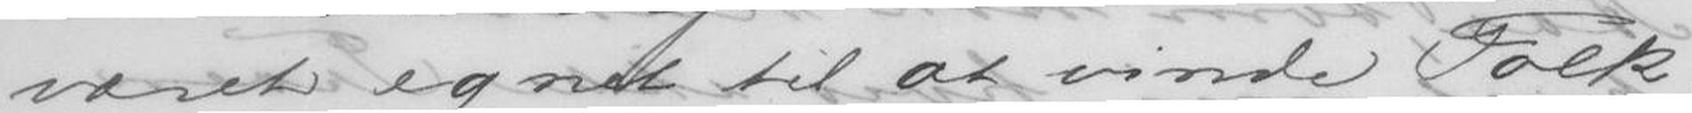været egnet til at vinde Folk,
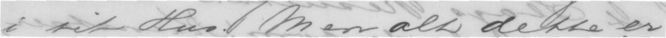i sit Hus. Men alt dette er
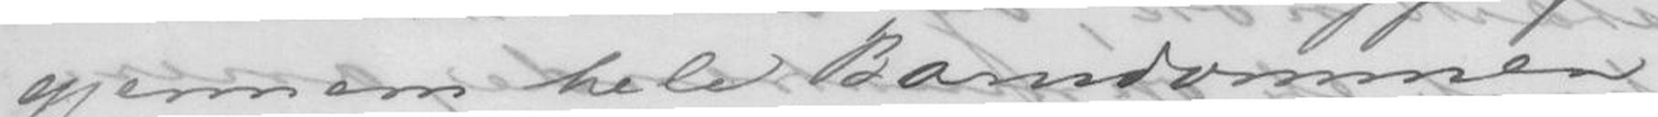gjennem hele Barndommen
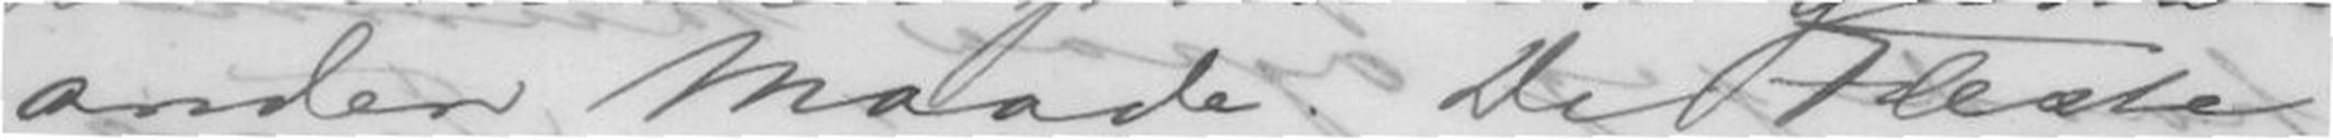anden Maade. De Fleste
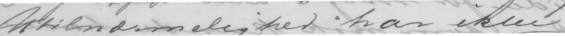Utilnærmelighed har ikke
